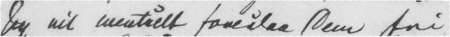Jeg vil trielt foreslaa Dem fri
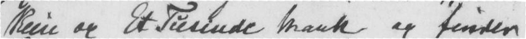Reise og Et Tusinde mark og finder
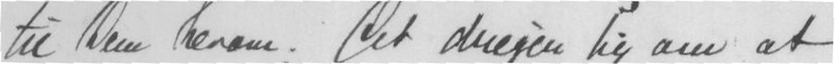til Dem herom. Det drejer Sig om at
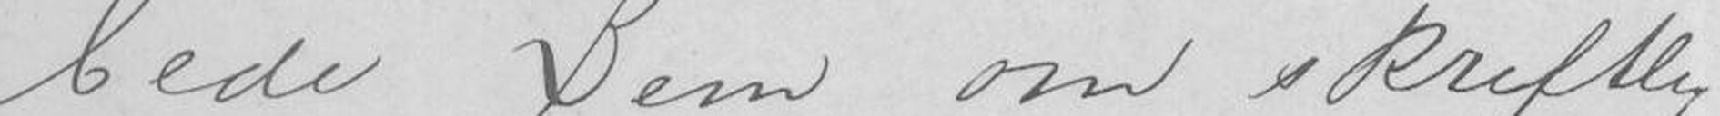bede Dem om skriftelig
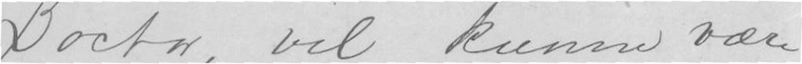Doctor, vil kunne være
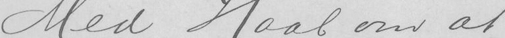Med Haab om at
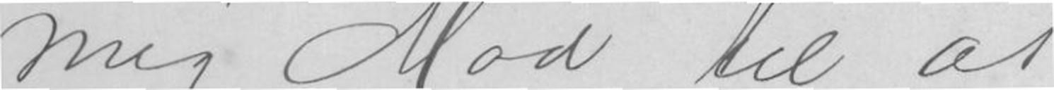mig Mod til at
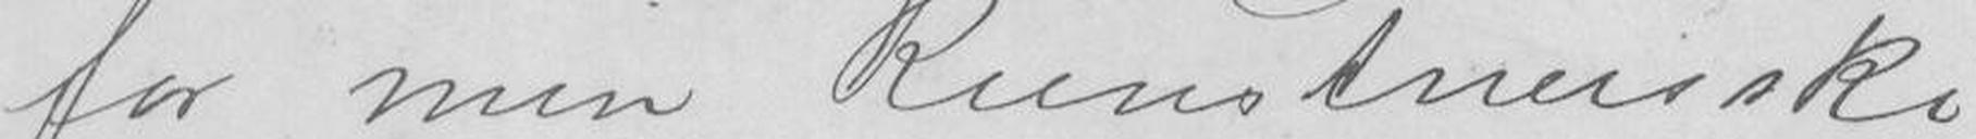for min kunstneriske
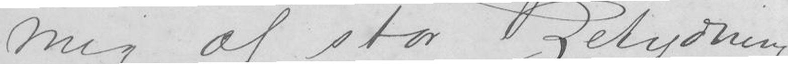mig af stor Betydning
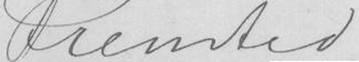Fremtid.-
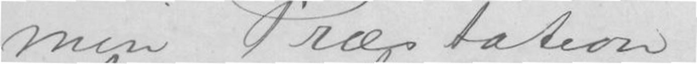min Prestation -
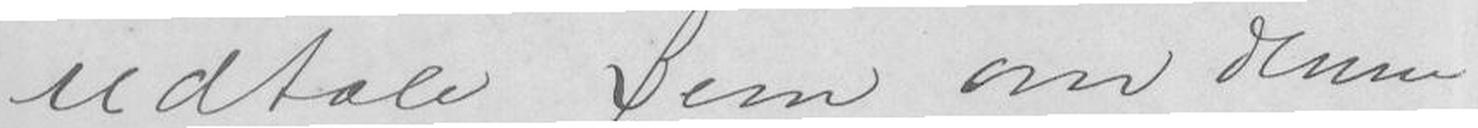udtale Dem om denne
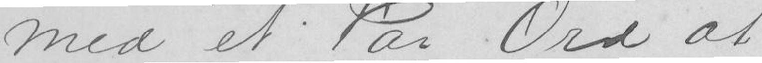med et Par Ord at
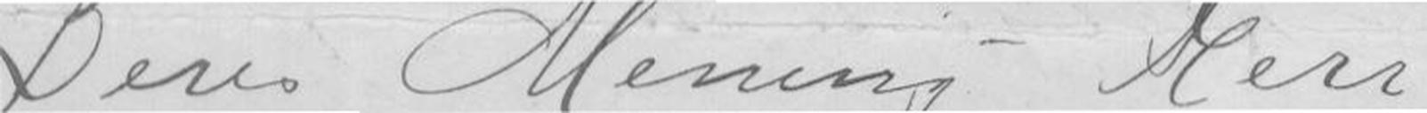Deres Mening Herr
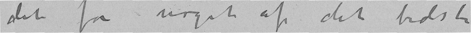det for noget af det bedste
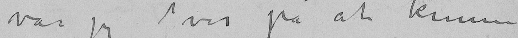var jeg vis på at kunne
